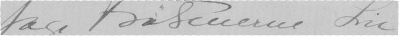tage Frøkenerne Lie
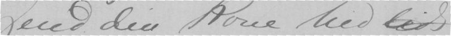send din Kone hid lidt
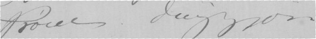Prøve. Din Bjørn.
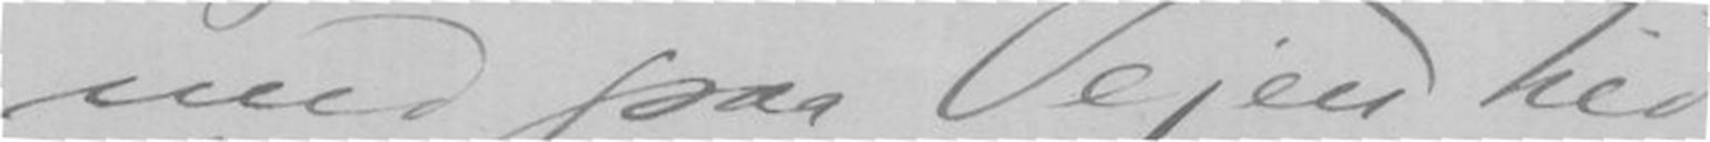med paa Vejen hid.
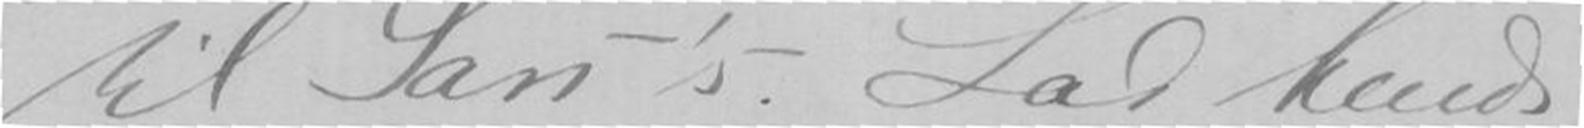til Sars's. Lad hende
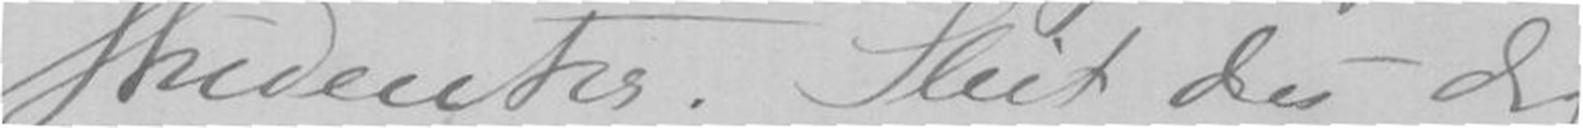Studenter. Slut du dig
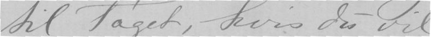til Toget, hvis du vil,
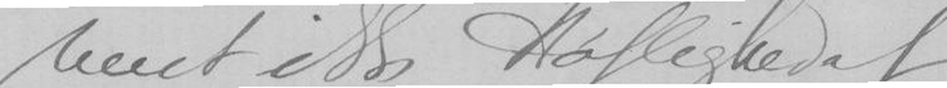Vent ikke Høflighed af
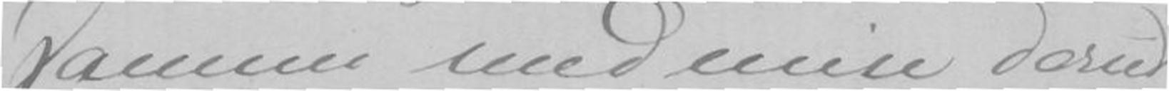sammen med min derud
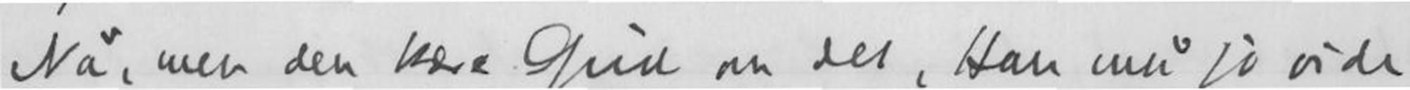Nå, mer den kære Gud om det, Han må jo vide
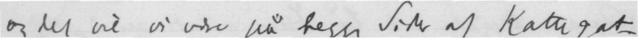og det vil vi være på begge Sider af Kattegat -
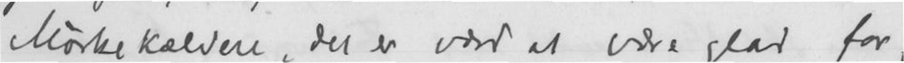Mørkekældere, det er værd at være glad for,
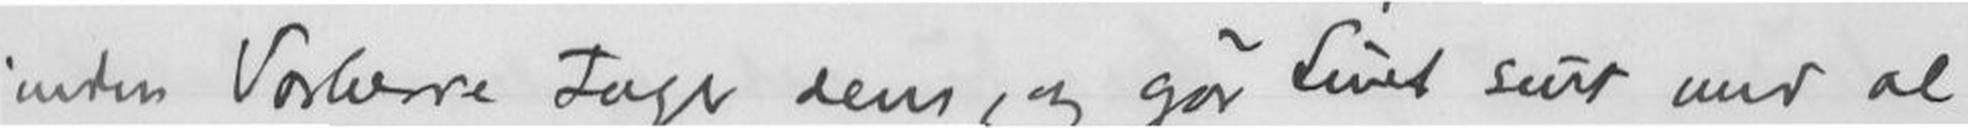inden Vorherre tager dem, og gør Livet surt med al
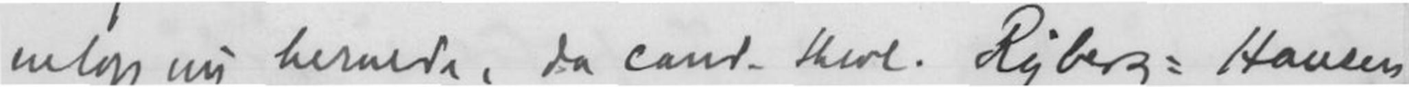netop nu hernede, da cand.theol. Ryberg=Hansen
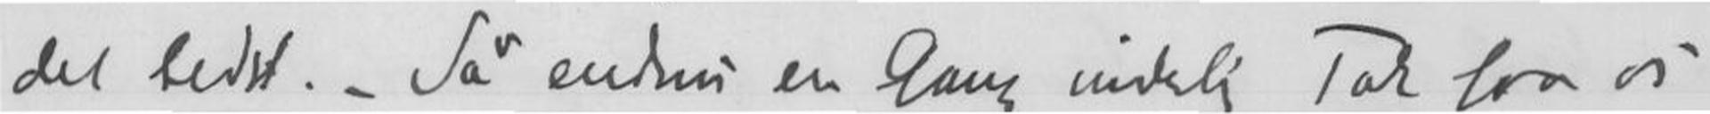det bedst. - Så endnu en Gang inderlig Tak for os
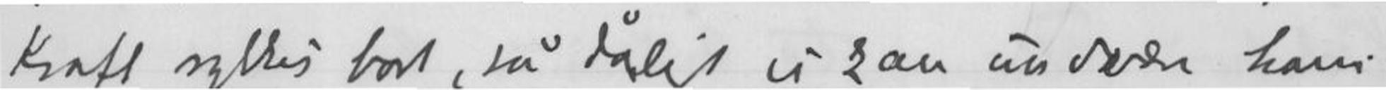kraft rykkes bort, så dårligt vi kan unvære ham.
